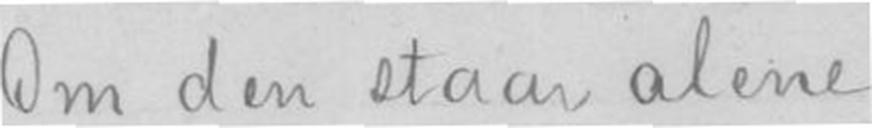Om den staar alene
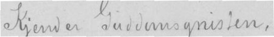Kjender Guddomsgnisten,
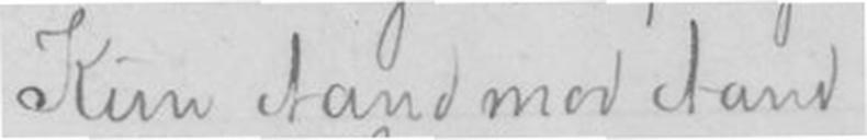Kun Aand mod Aand
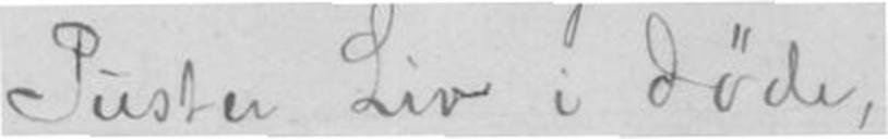Puster Liv i døde,
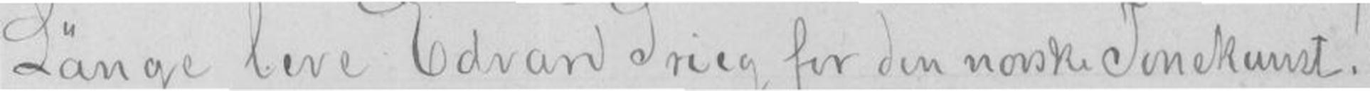Länge leve Edvard Grieg for den norske Tonekunst!
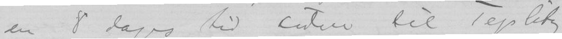en 8 dagers tid siden til Teplitz,
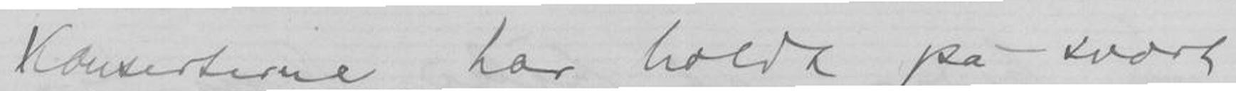Konserterne har holdt på - svært
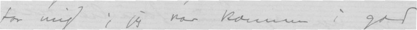for mig; jeg var kommen i god
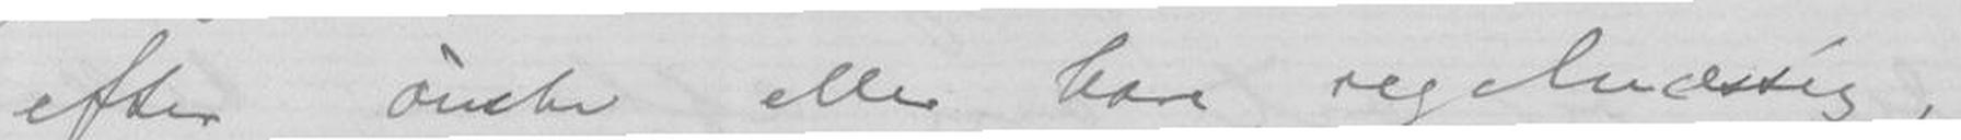efter ønske eller bare regelmæssig,
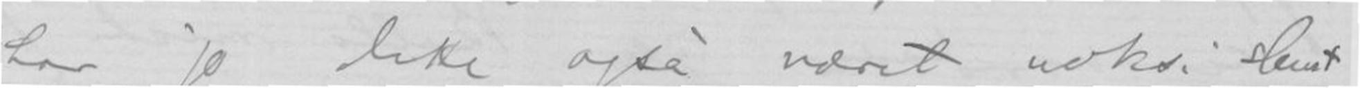har jo dette også været nokså slemt
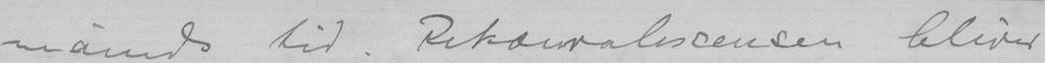måneds tid. Rekonvaliscensen bliver
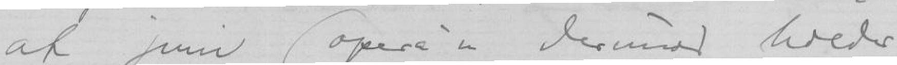af juni (opera'n derimod holder
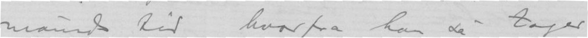måneds tid, hvorfra han så tager
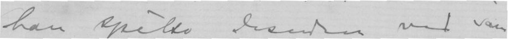(han spilte desuden ved sam-
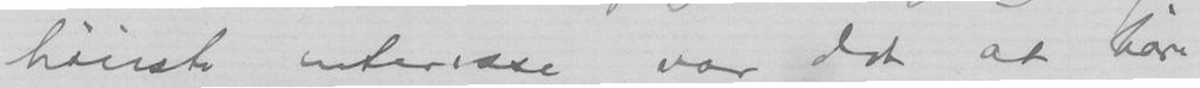høieste intresse var det at høre
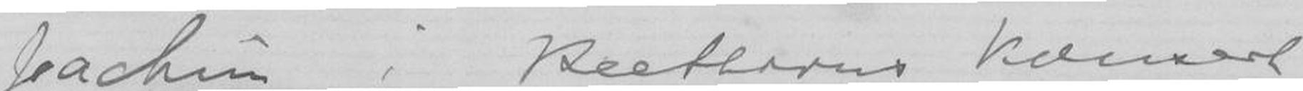Joachim i Beethovens komsert
